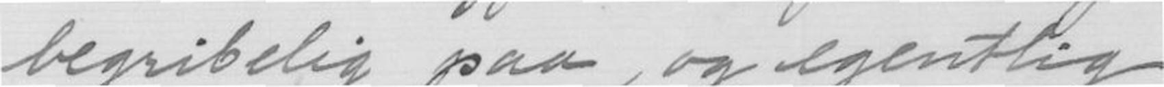begribelig paa, og egentlig
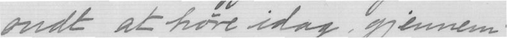ondt at høre idag, gjennom
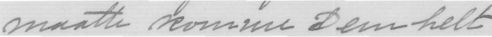maatte komme Dem helt,
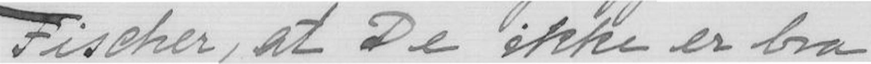Fischer, at De ikke er bra
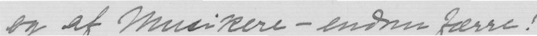og af Musikere - endnu færre!
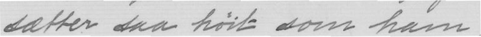sætter saa høit som ham,
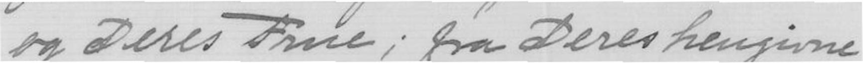og Deres Frue; fra Deres hengivne
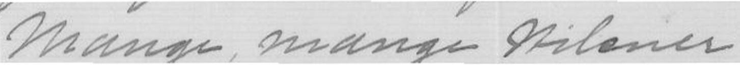Mange, mange Hilsener
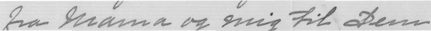fra Mama og mig til Dem
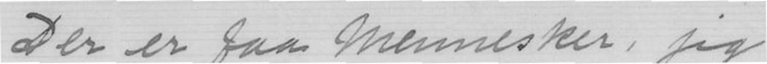Der er faa mennesker, jeg
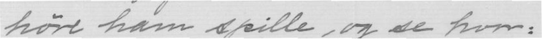behøver ham spille, og se hvor-
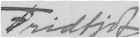Fridtjof
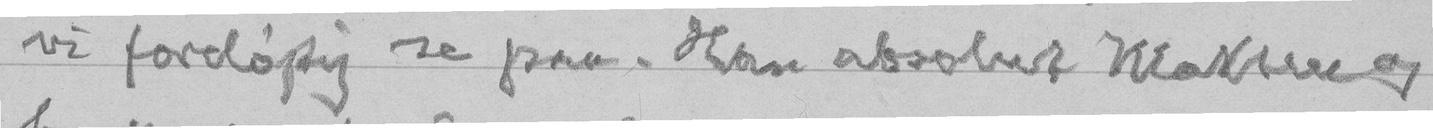vi foreløpig se paa. Han absolut Makten og
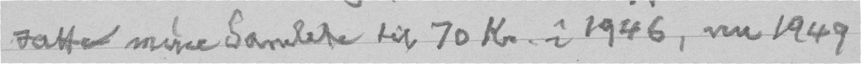satte mine Samlede til 70 Kr. i 1946, nu 1949
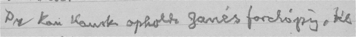Du kan kanske opholde Janés foreløpig, til
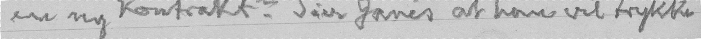en ny Kontrakt? Sier Janés at han vil trykke
